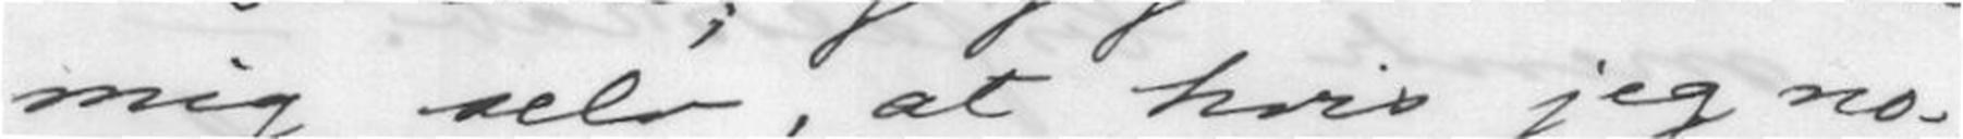mig selv, at hvis jeg no-
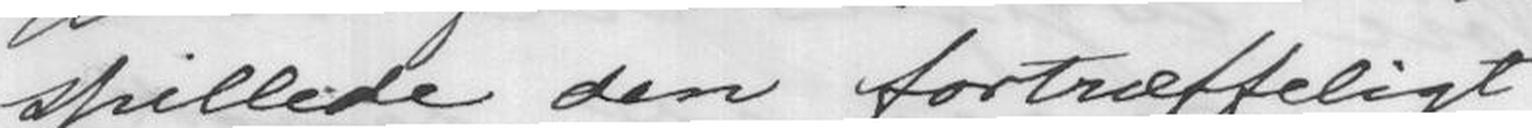spillede den fortræffeligt
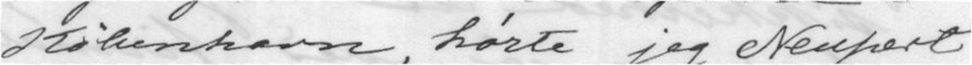København, hørte jeg Neupert
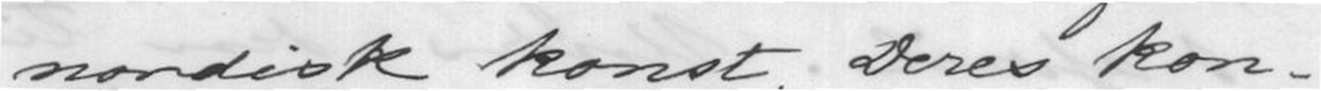nordisk konst, Deres kon-
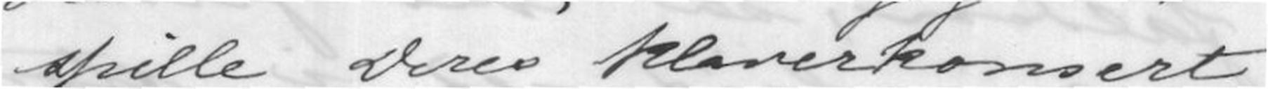spille Deres Klaverkonsert
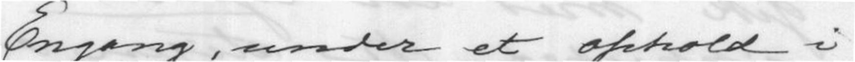Engang under et ophold i
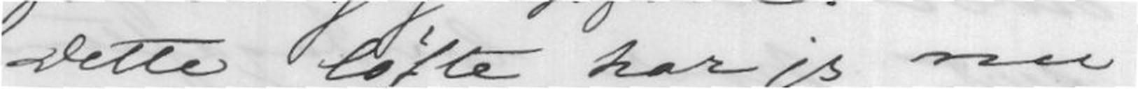Dette løfte har jeg nu
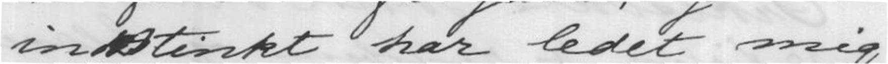indstinkt har ledet mig
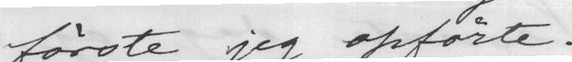første jeg opførte.
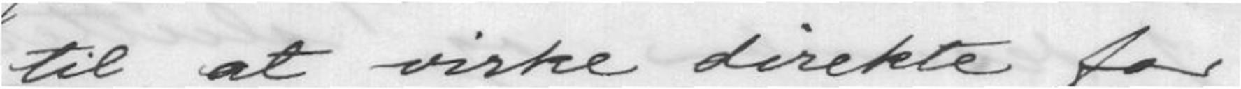til at virke direkte for
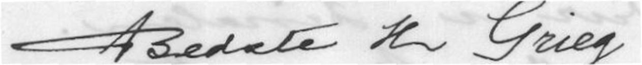Bedste Hr. Grieg!
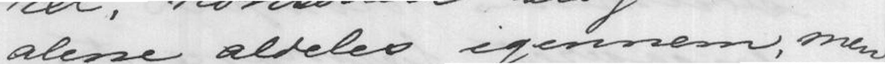alene aldeles igjennem, men
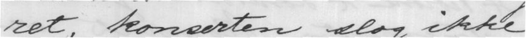ret, konserten slog ikke
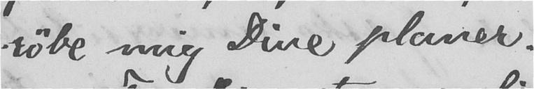røbe mig Dine planer.
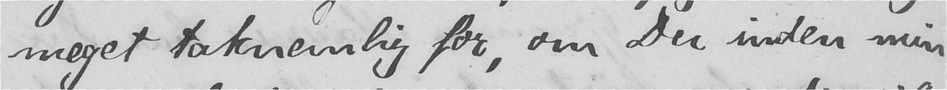meget taknemlig for, om De inden min
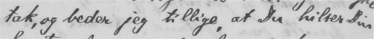tak, og beder jeg tillige, at Du hilser Din
